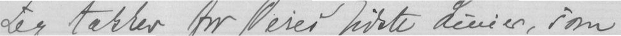Jeg takker for Deres sidste Linier, som
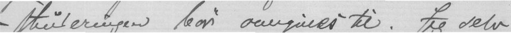- bør overgives til. Jeg selv
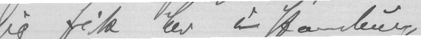jeg fik her i Hamburg.
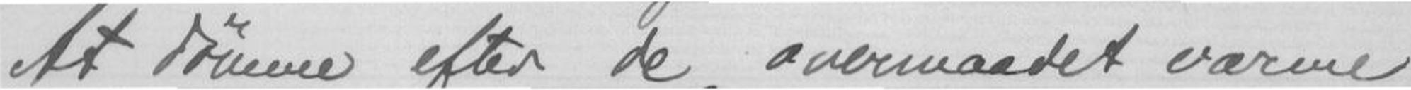At efter de overmaadet varme
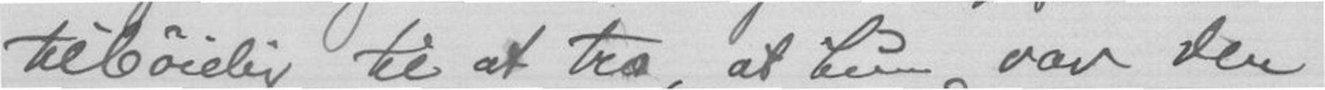tilbøielig til at tro, at hun var den
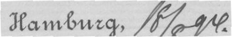Hamburg, 18/8 - 94.
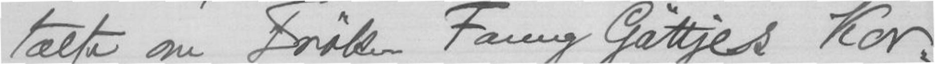talte om Frøken Fanny Gattjes Kor-
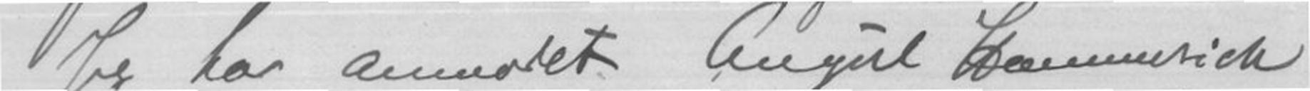Jeg har anmodet Angul Hammerick
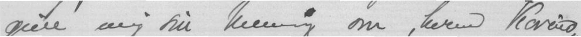give sig nu hermed om, Korind-
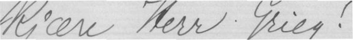Kjære Herr Grieg !
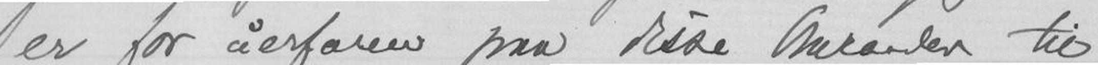er for uerfaren paa disse Omraader til
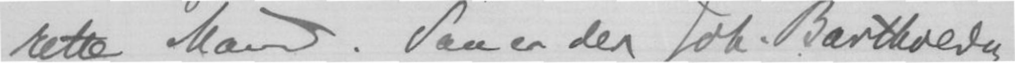rette Mand. Saa er der Joh. Bartholdy
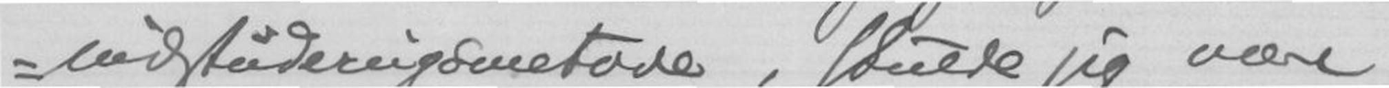indstuderingsmetode, skulde jeg være
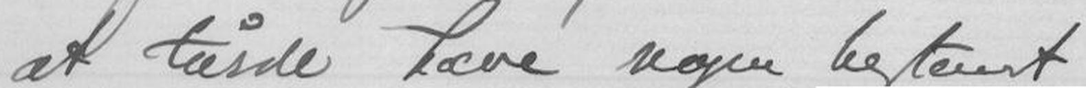at kunde love nogen bestemt
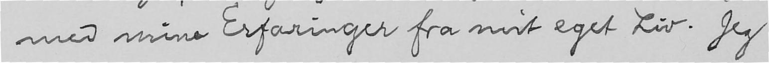med mine Erfaringer fra mit eget Liv. Jeg
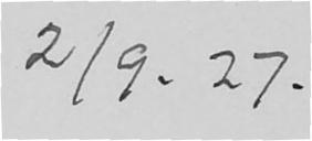2/9. 27.
